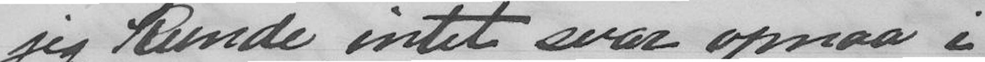jeg kunde intet svar opnaa i
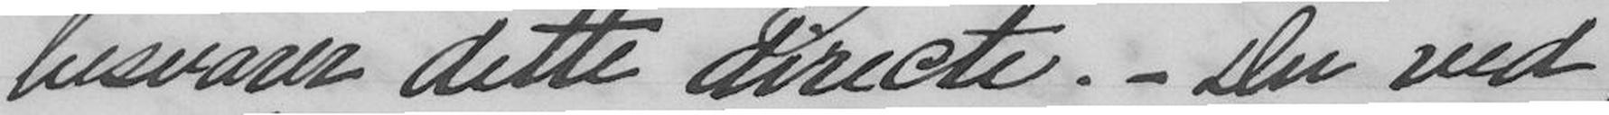besvarer dette directe. - Du ved,
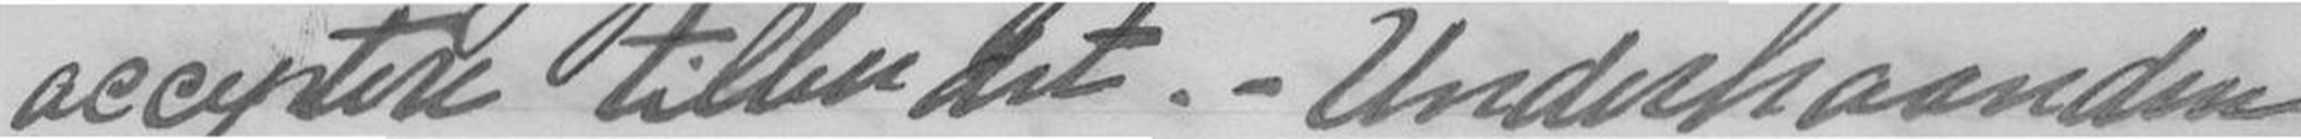acceptere tilbudet. - Underhaanden
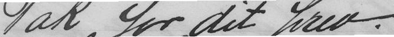Tak for dit brev.
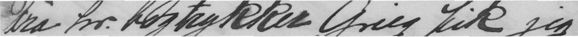Fra hr. bogtrykker Grieg fik jeg
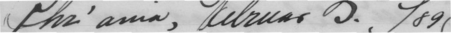Chr'ania, Februar 5. 189
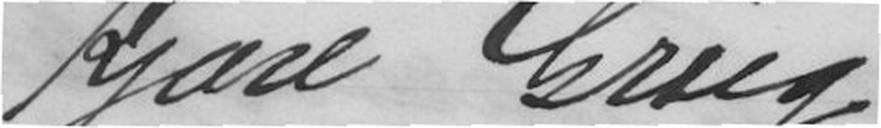Kjære Grieg
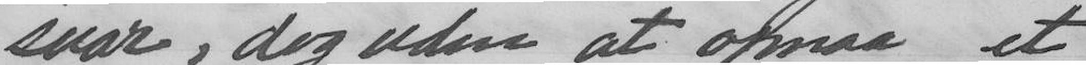svar, dog uden at opnaa et
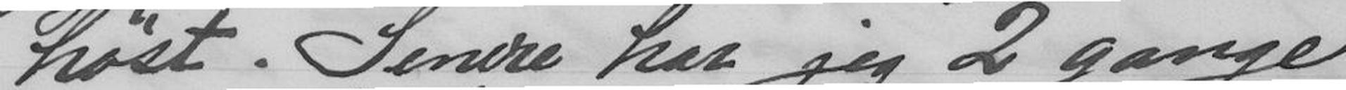høst. Senere har jeg 2 gange
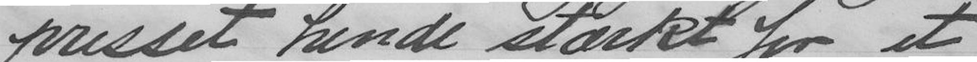presset hende stærkt for et
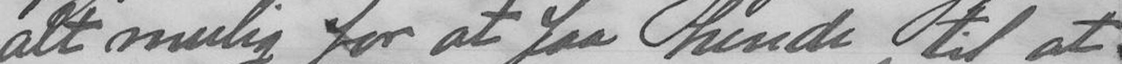alt mulig for at faa hende til at
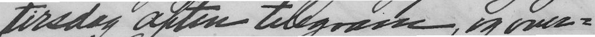tirsdag aften telegram, og over-
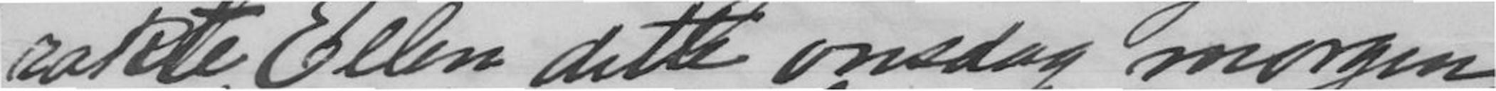rakte Ellen dette onsdag morgen,
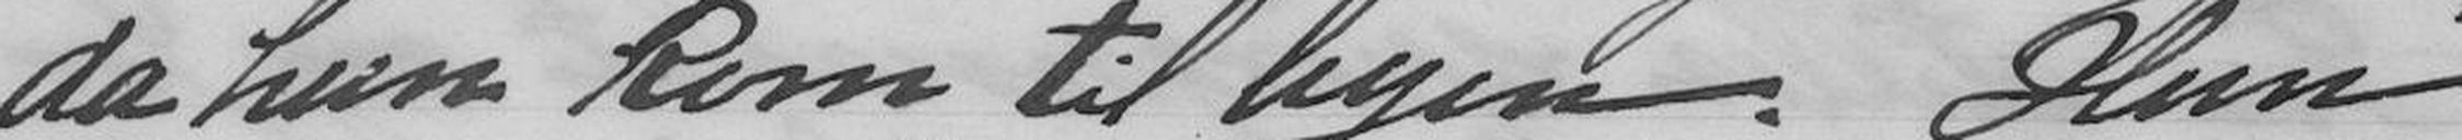da hun kom til byen. Hun
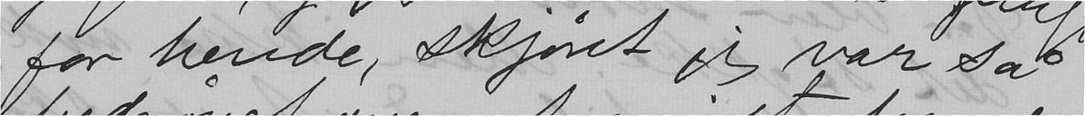for hende, skjønt jeg var så
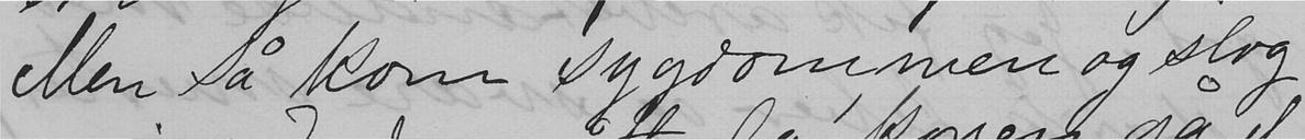Men så kom sygdommen og slog
